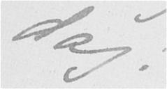dag.
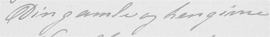Din gamle og hengivne
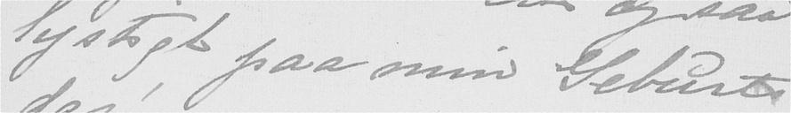lystigt paa min Geburts-
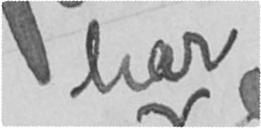har
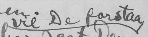vil De forstaa
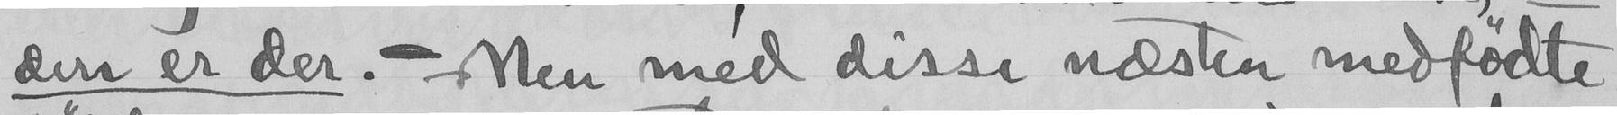den er der. - Men med disse næsten medfødte
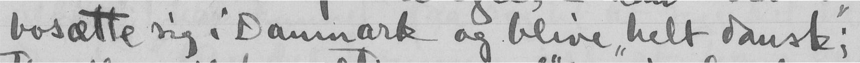bosætte sig i Dammark og blive "helt dansk";
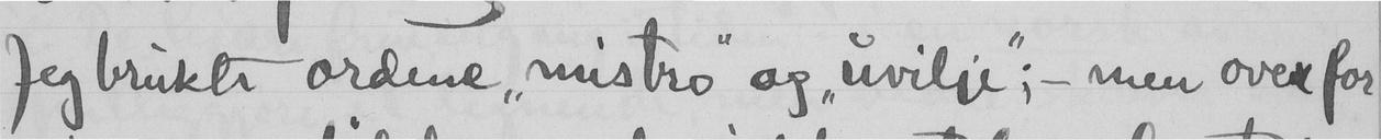Jeg brukte ordene "mistro" og "uvilje"; - men overfor
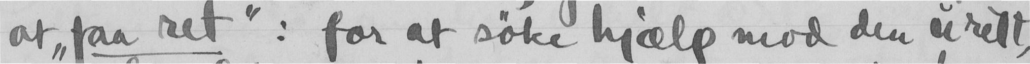at "faa ret": for at søke hjælp mod den urett,
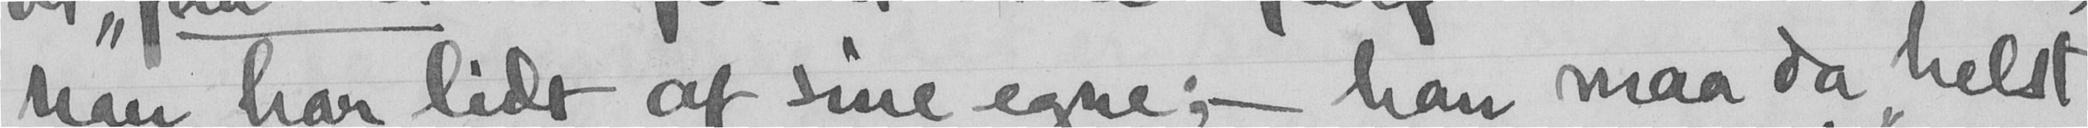han har lidt af sine egne; - han maa da helst
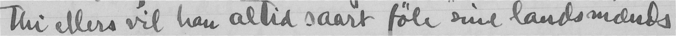thi ellers vil han altid saart føle sine landsmænds
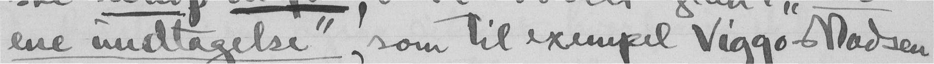ene undtagelse", som til exempel Viggo Madsen
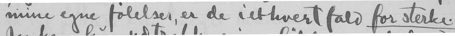mine egne følelser, er de iethvertfald for sterke.
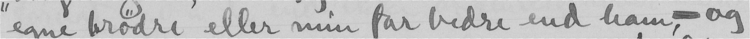egne brødre eller min far bedre end ham- og
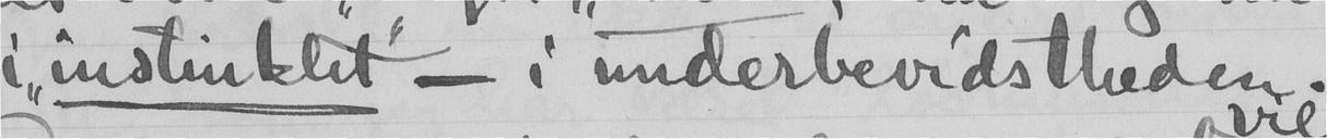i "instinktet" - i underbevidstheden.
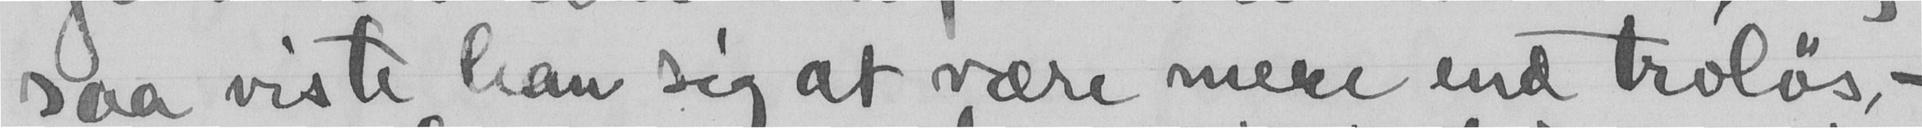saa viste han sig at være mere end troløs, -
